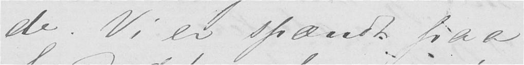de. Vi er spændt paa
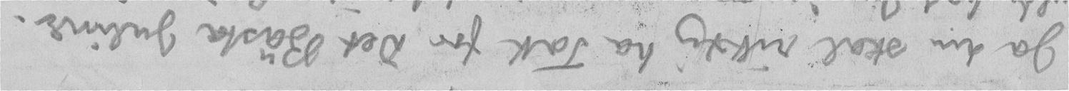Ja du skal riktig ha Tak for Det Bästa Julinr.
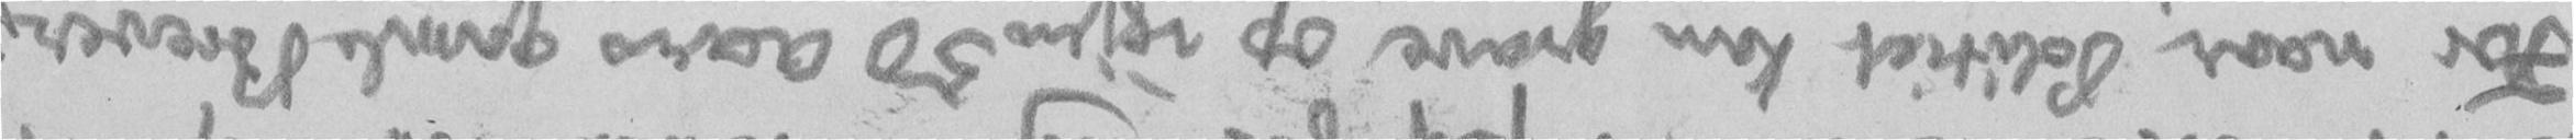For naar Politiet kan grave op igjen 50 Aars gamle Brever
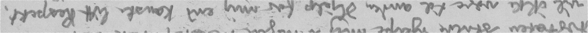vil ikke være til anden Hjelp for mig end kanske litt Respekt.
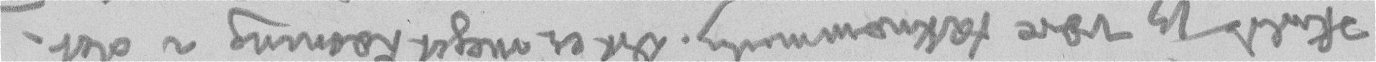skulde jeg være taknemmelig. Det er meget Læsning i det.
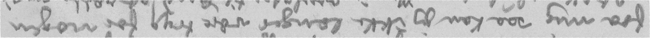fra mig saa kan jeg ikke længer være tryg for nogen
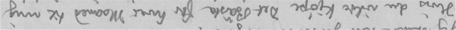Hvis du vilde kjøpe Det Bästa for hver Maaned til mig
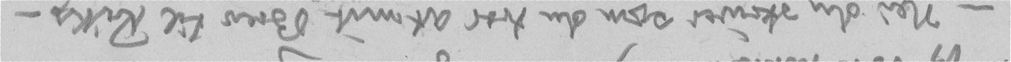- Nei du skriver som du tror at mit Brev til Riks-
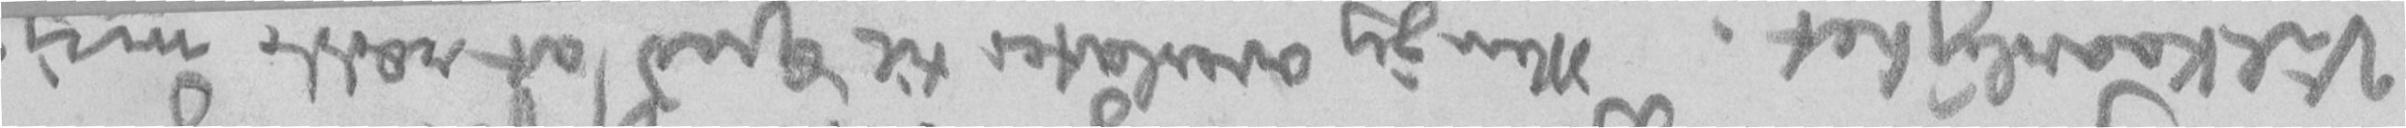Vilkaarlighet. Men jeg overlater til Gud at rædde mig
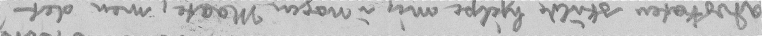advokaten skulde hjelpe mig i nogen Maate, men det
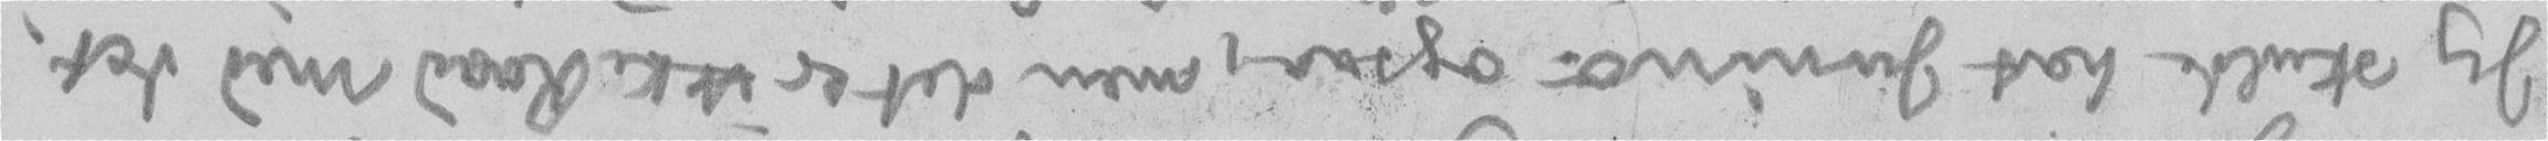Jeg skulde hat Junino. ogsaa, men det er ikke Raad med det.
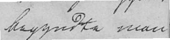begyndte man
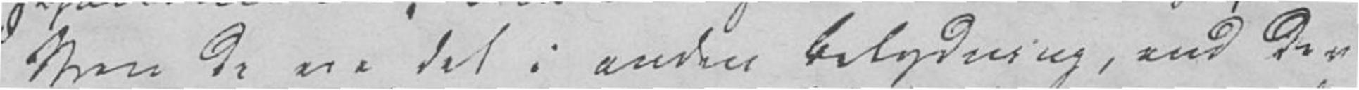Men de ere det i anden Betydning, end den
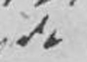de
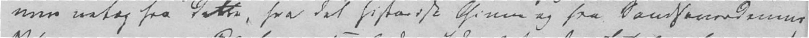men netop fra dette, fra det historisk Givne og fra Sandseverdenens
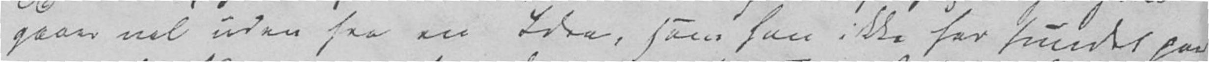gaaer vel uden fra en Idee, som han ikke har fundet paa
